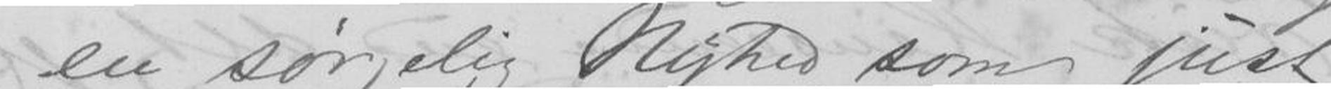en sørgelig Nyhed som just
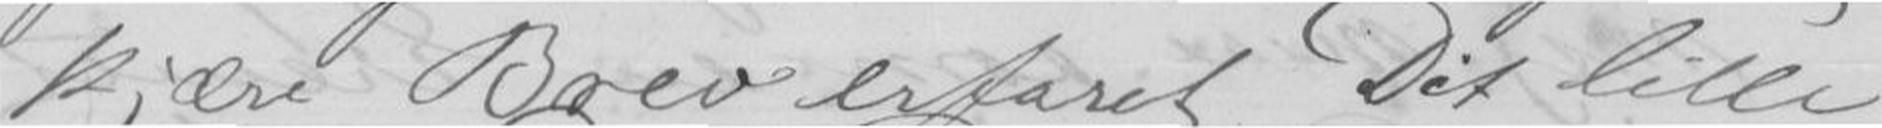kjære Brev erfaret Det lille
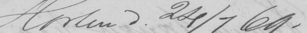Horten 24/7.69
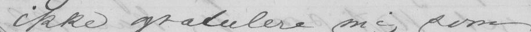ikke gratulere mig som
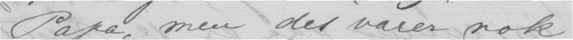Papa, men det varer nok
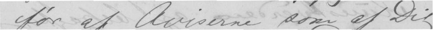før af Aviserne som af Dit
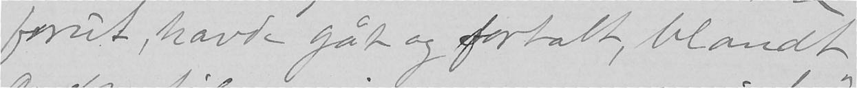forut, havde gåt og fortalt, blandt
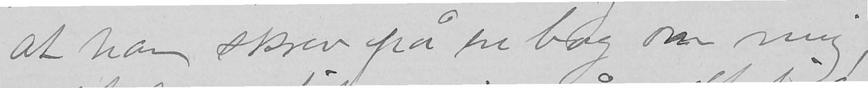at han skrev på en bog om mig
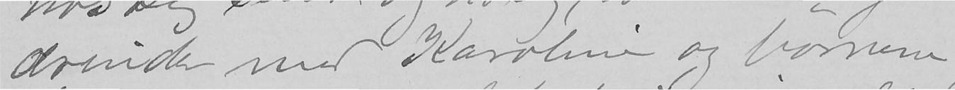derinde med Karoline og børnene,
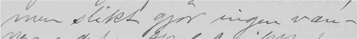men slikt gjør ingen væn-
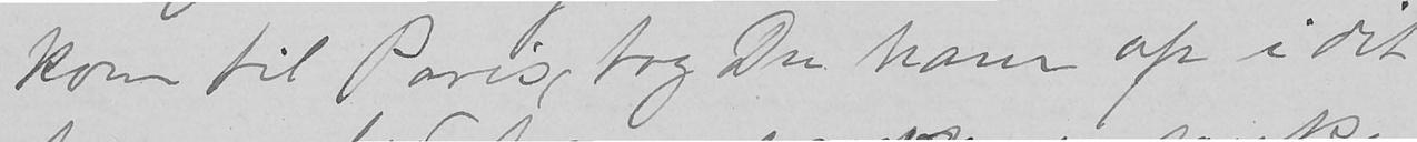kom til Paris, tog Du ham op i dit
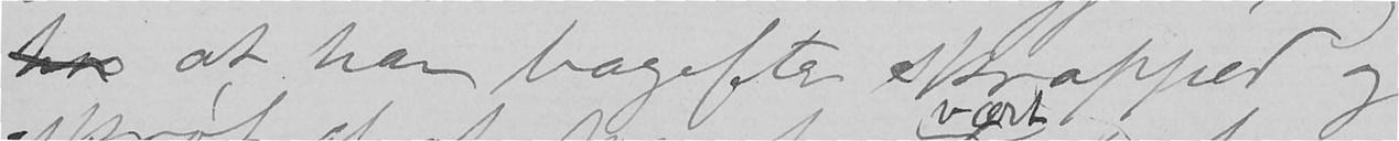hos at han bagefter skræpped og
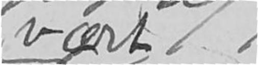vært
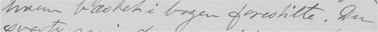hvem bæstet i bogen forestilte. Du
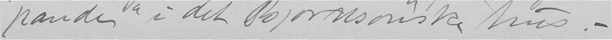pande" i det Bjørnsonske hus.-
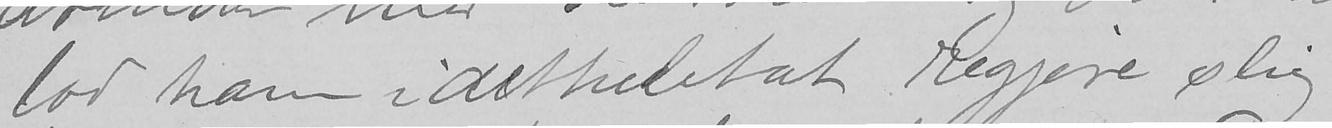lod ham idetheletat regjere slig
